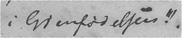i Gjenfødelsen."
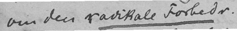om den radikale Forbedr.
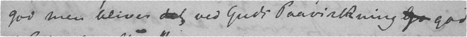god men bliver det ved Guds Paavirkning god
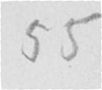55
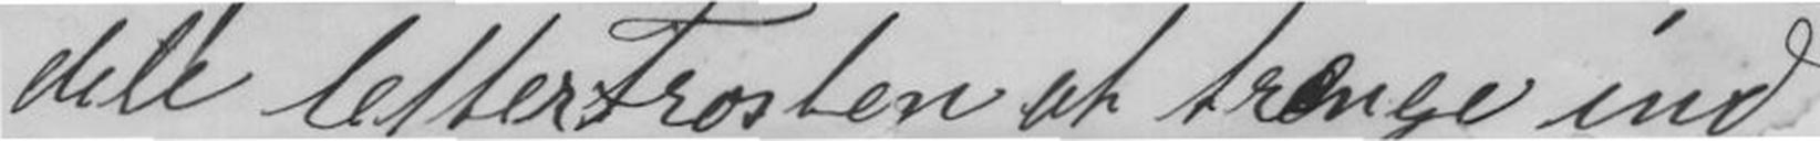dele letter Frosten at trænge ind
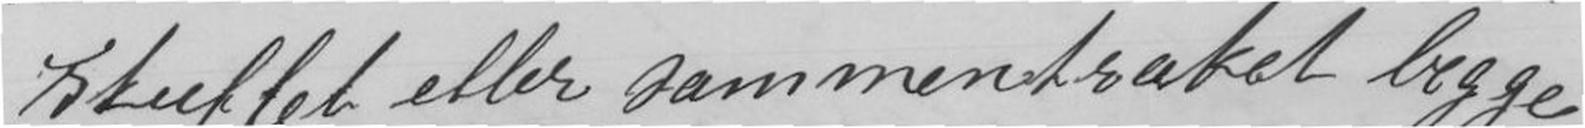skuflet eller sammentraket begge
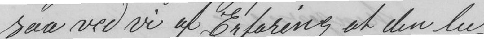saa ved vi af Erfaring at den lu-
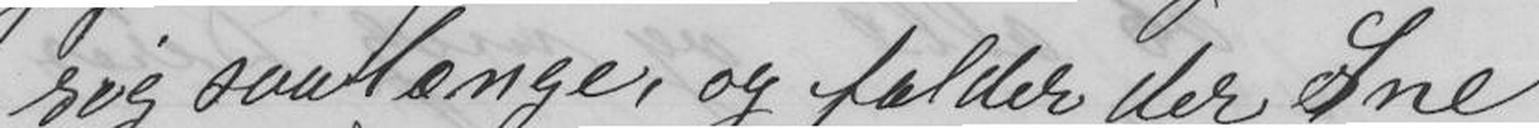sig saalænge, og falder der Sne
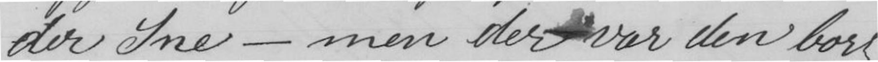der Sne - men der var den bort
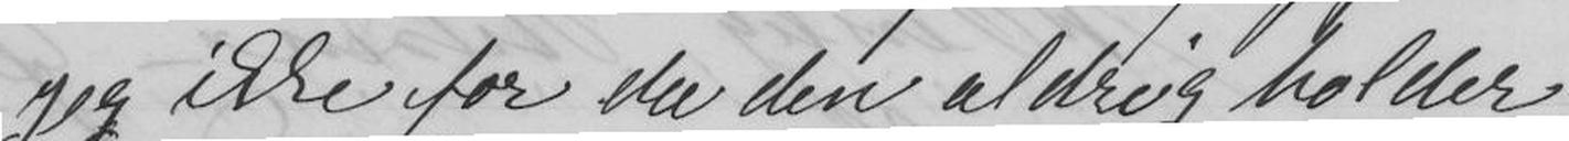jeg ikke for da den aldrig holder
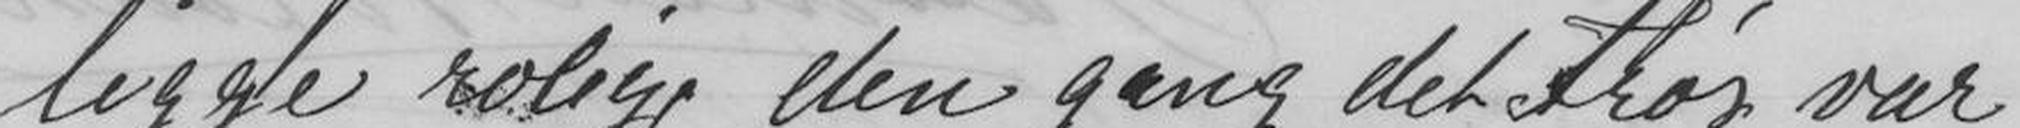ligge rolig, den gang det Frøs var
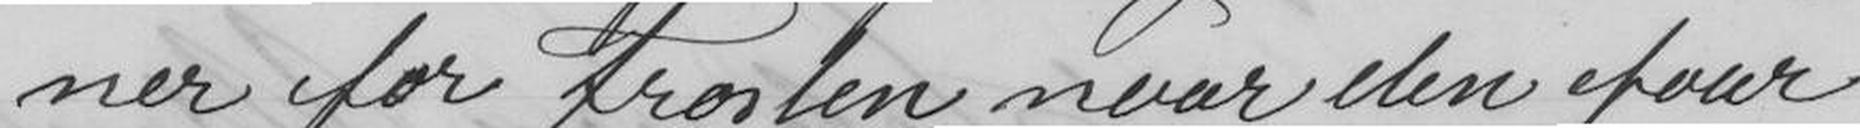ner for Frosten naar den faar
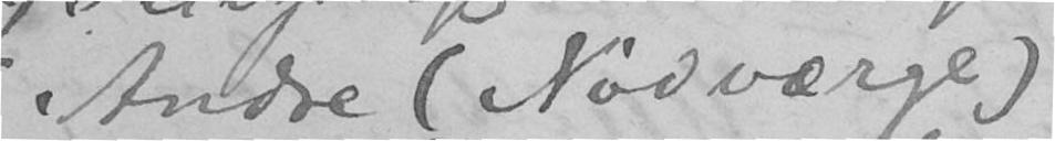Andre (Nødværge).
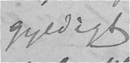gyldigt.
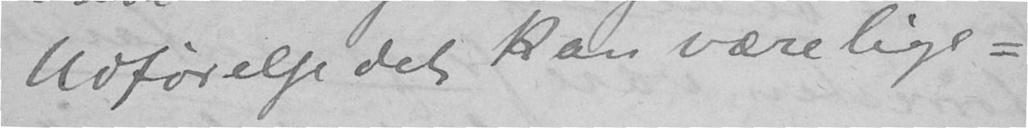Udførelse det kan være lige-
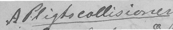A Pligtscollisioner
